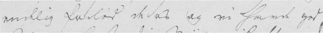endelig forlod de os og vi havde god
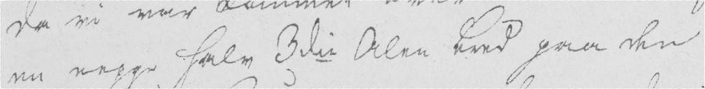en neppe halv 3die Alen bred paa den
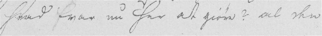hvad var nu her at giøre? al den
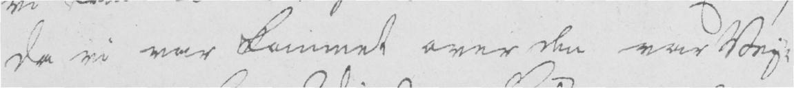da vi var kommet over den var Vey-
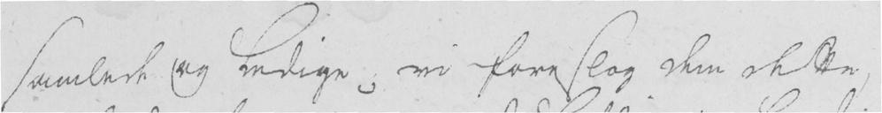samlede og ledige, vi foreslog dem dette,
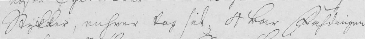Stykker, enhver tog sit; 4 bar Faldingen
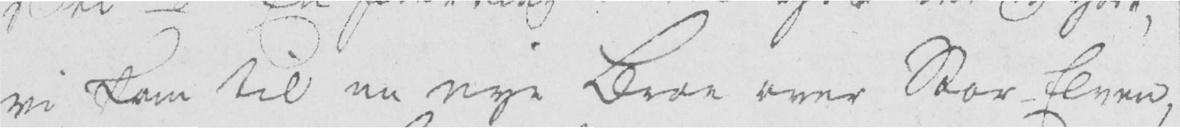vi kom til en nye Broe over Stor-Elven,
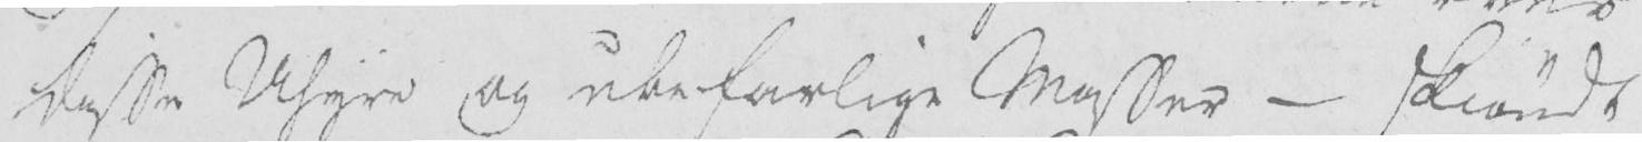disse Uhyre og ubefarlige Masser - skiøndt
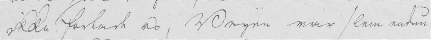ikke forlade os, Veyen var slem endnu
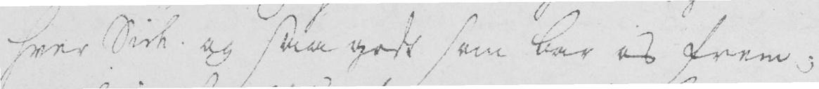hver Side og saa godt som bar os frem;
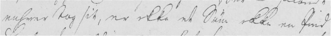enhver tog sit, er ikke et Søm ikke en Pind
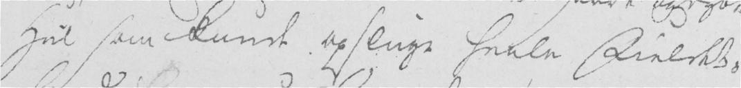Hul som kunde opsluge heele Fieldet,
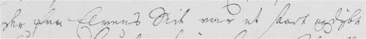der fra Elvens Side var et stort og dybt
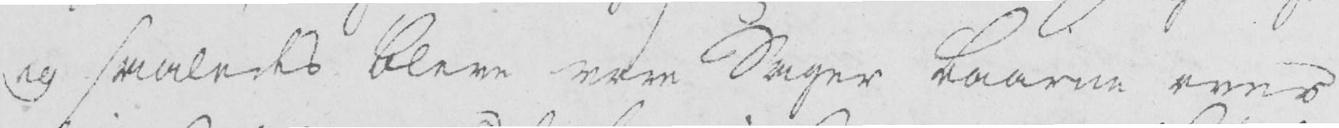og saaledes bleve vore Sager baarne over
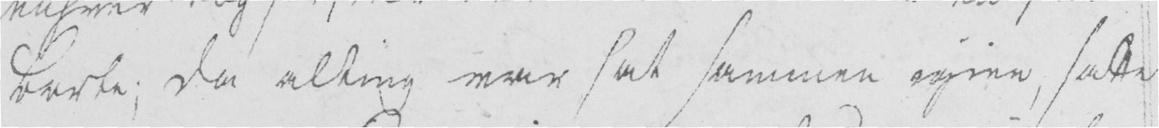borte; da alting var sat sammen igien, satte
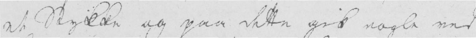et Stykke og paa dette gik nogle ved
